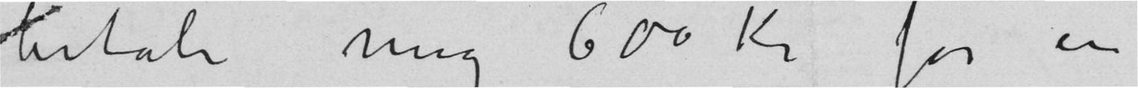betale mig 600 kr for en
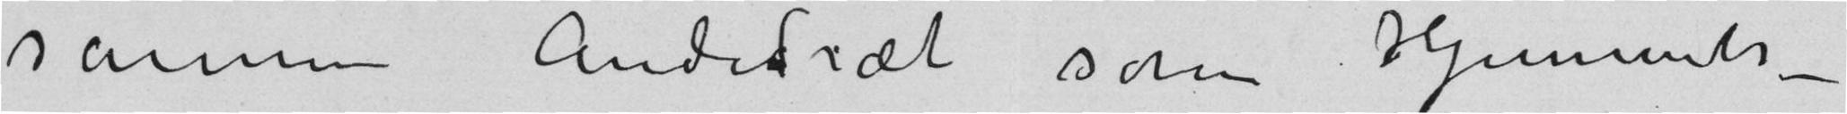samme Åndedræt som Hjemmets –
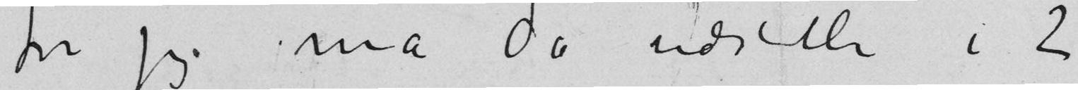for jeg må da udstille i 2
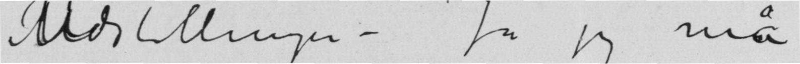Udstillingen – Ja jeg må
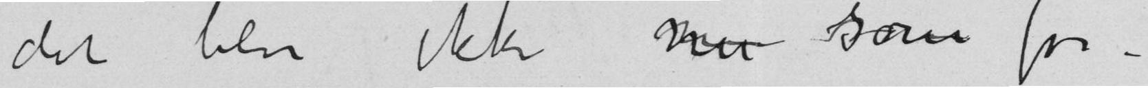det blir ikke nu som før –
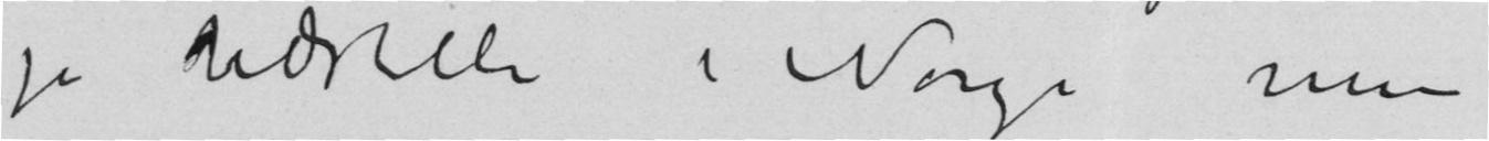jo udstille i Norge men
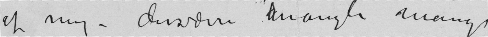af mig – desværre mangler mange
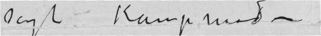sagt Kampmod –
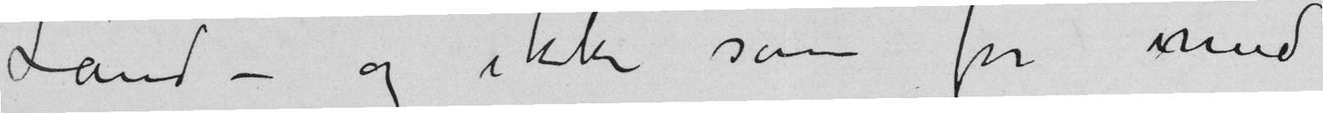Land – og ikke som før med
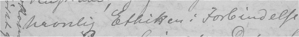navnlig Ethiken i Forbindelse
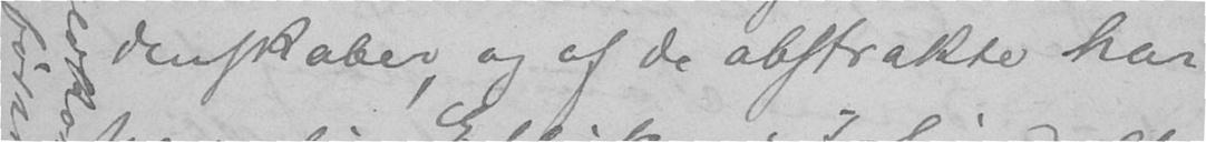denskaber, og af de abstrakte har
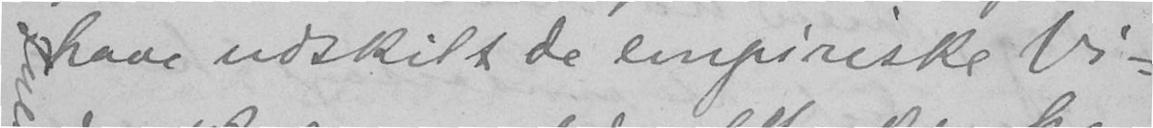have udskilt de empiriske Vi-
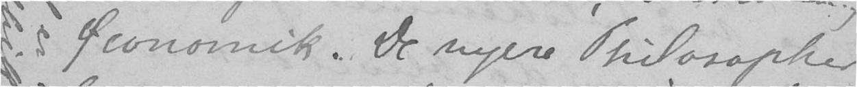Economik. De nyere Philosopher
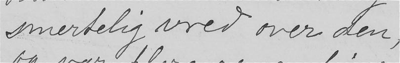smertelig vred over den,
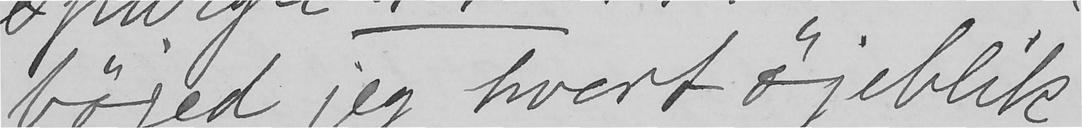bøjed jeg hvert øjeblik
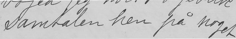samtalen hen på noget
